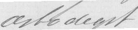ærbødigst
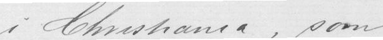i Christiania, som
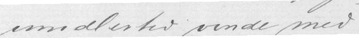imiglertid vinde med
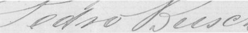Pedro Busch
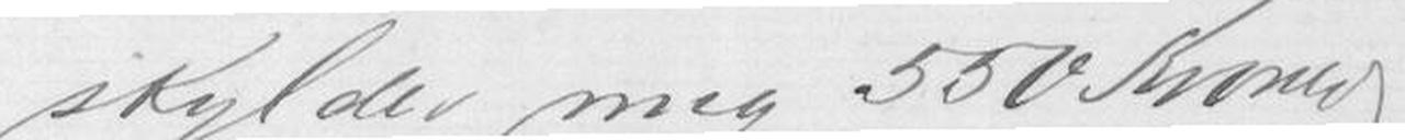skylder mig 550 Kroner.
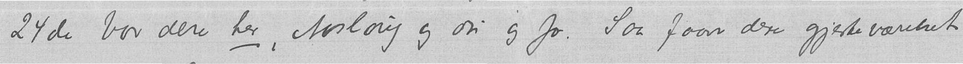24de bor dere her, Aaslaug og du og Jo. Saa faar dere gjesteværelset
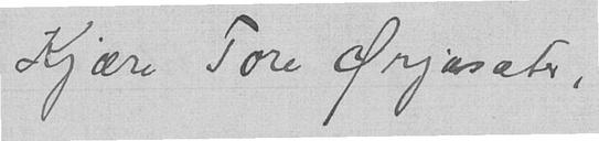Kjære Tore Ørjasæter,
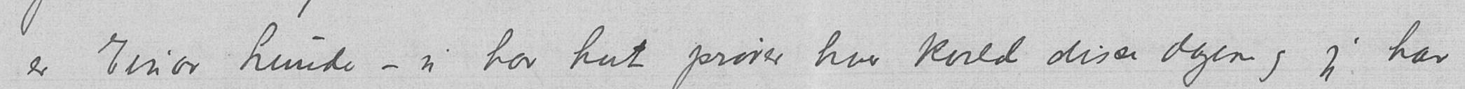er Einar Lunde – vi har hat prøver hver kveld disse dagene og jeg har
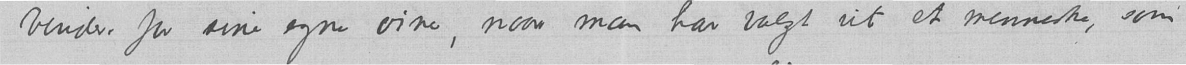binder for sine egne øine, naar man har valgt ut et menneske, som
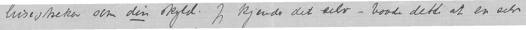lusestreker som din skyld. Jeg kjender det selv – baade dette at en selv
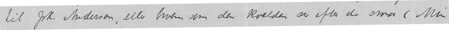til frk. Andersen, eller hvem som den kvelden ser efter de smaa (Min
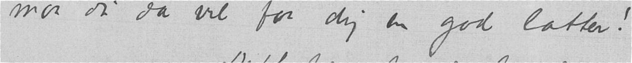maa du da vel faa dig en god latter!
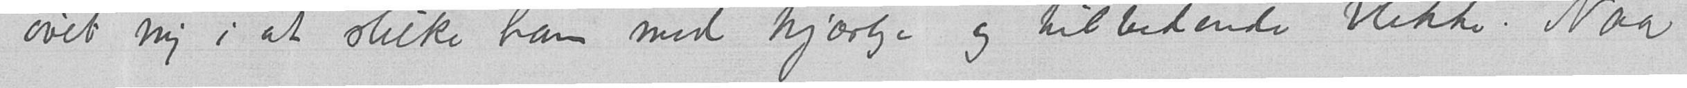øvet mig i at sluke ham med kjærlige og tilbedende blikke. Naa
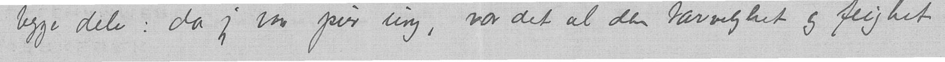begge dele: da jeg var pur ung, var det al den tarvelighet og feighet
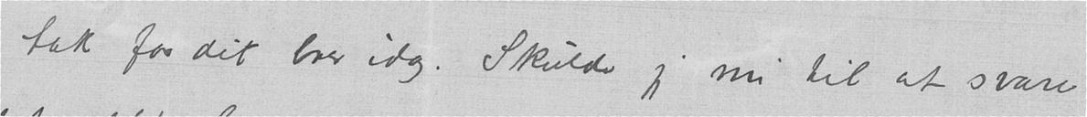tak for dit brev idag. Skulde jeg nu til at svare
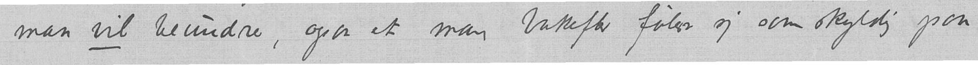man vil beundre, ogsaa at man bakefter føler sig som skyldig paa
